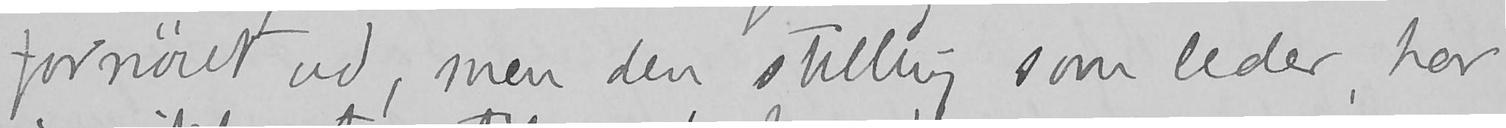fornøiet ud, men den stilling som leder, har
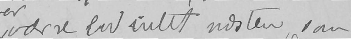værre end intet næsten som
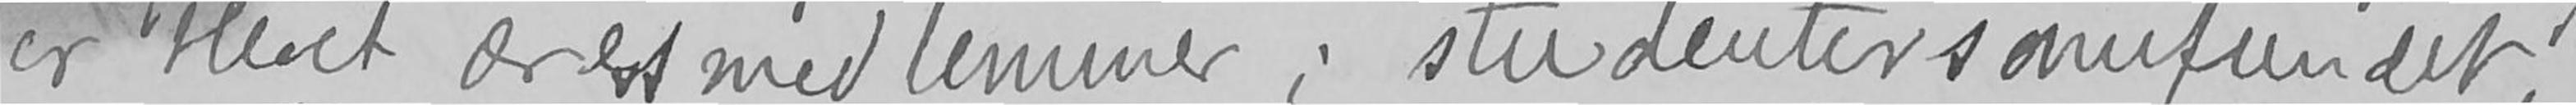er blevet ærede medlemmer i studentersamfundet,
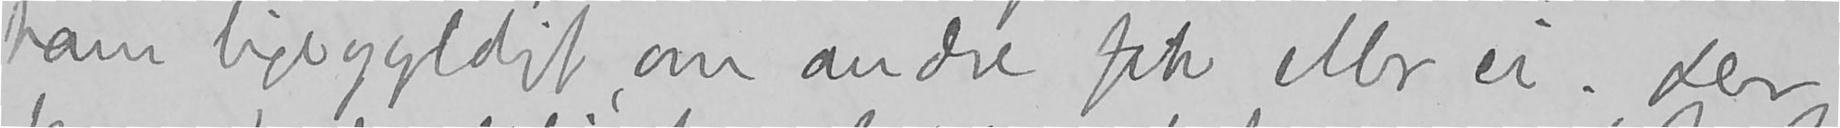ham ligegyldigt om andre fik eller ei. Der
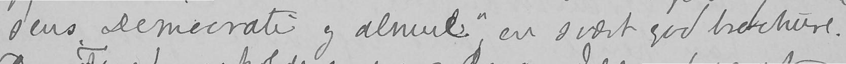sens Democrati og almue en svært god brochure.
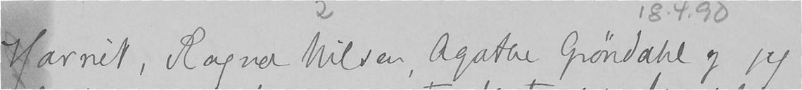Harriet, Ragna hilser, Agathe Grøndahl og jeg
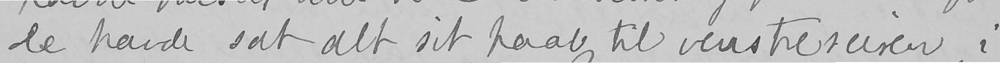De havde sat alt sit haab til venstreseiren i
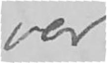var
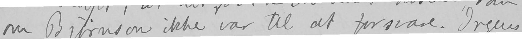om Bjørnson ikke var til at forsvare. Irgens
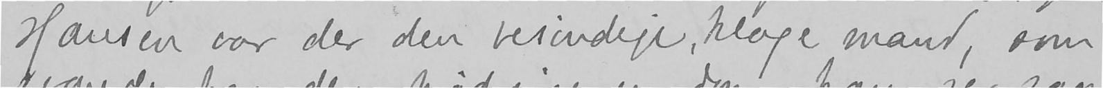Hansen var der den besindige, kloge mand, som
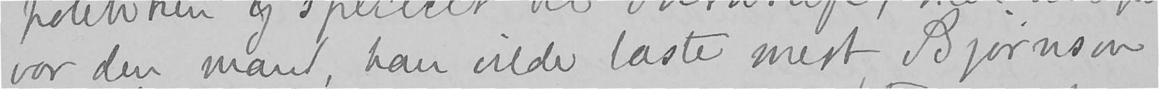var den mand, han vilde laste mest Bjørnson.
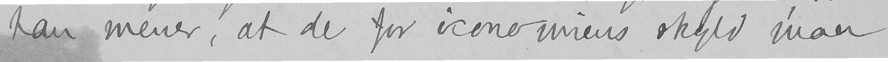han mener, at de for øconomiens skyld maa
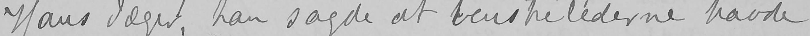Hans Jæger, han sagde at venstrelederne havde
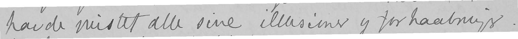havde mistet alle sine illusioner og forhaabninger.
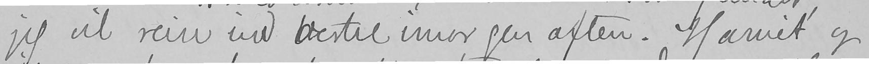jeg vil reise ind dertil imorgen aften. Harriet og
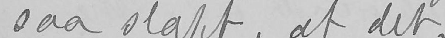saa slapt, at det
